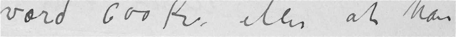værd 600 Kr. eller at han
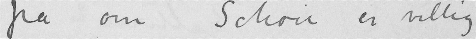på om Schou er villig
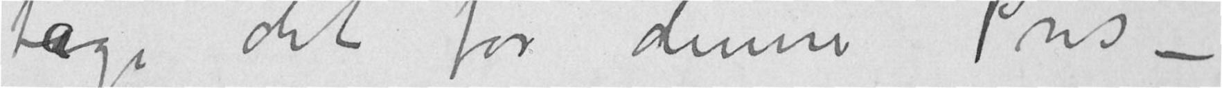tage det for denne Pris –
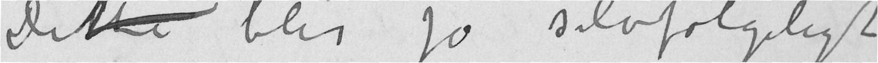Dette blir jo selvfølgeligt
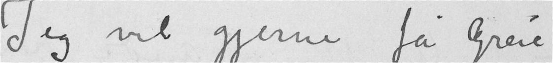Jeg vil gjerne få Greie
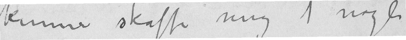kunne skaffe mig nogle
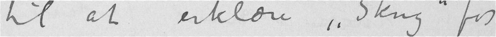til at erklære «Skrig» for
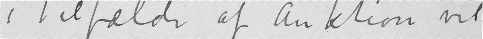i Tilfælde af Auktion vil
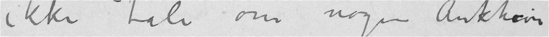ikke Tale om nogen Auktion
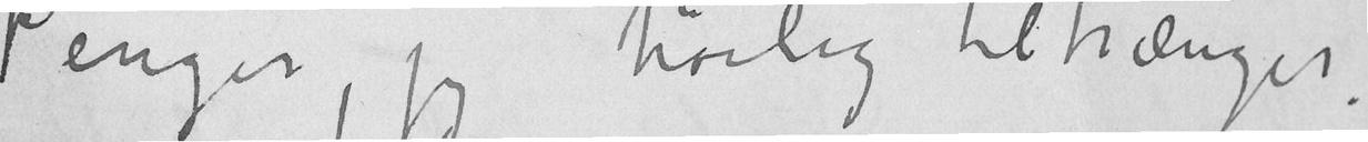Penger, jeg høilig tiltrænger.
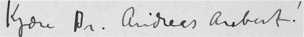Kjære Dr. Andreas Aubert!
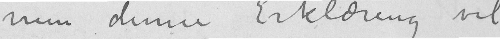men denne Erklæring vil
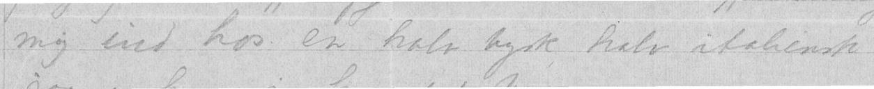mig ind hos en halv tysk halv italienske.
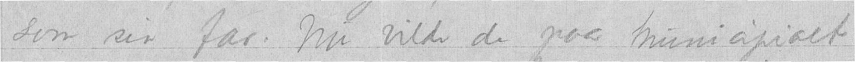som sin far. Nu vilde de naa Municipioet
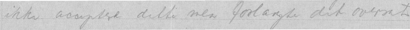ikke acceptere dette, men forlangte det oversat
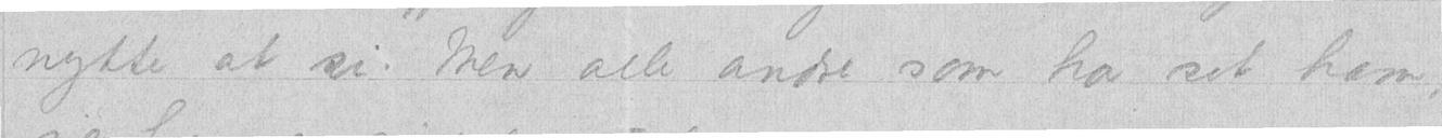nytte at si. Men alle andre som har set ham,
